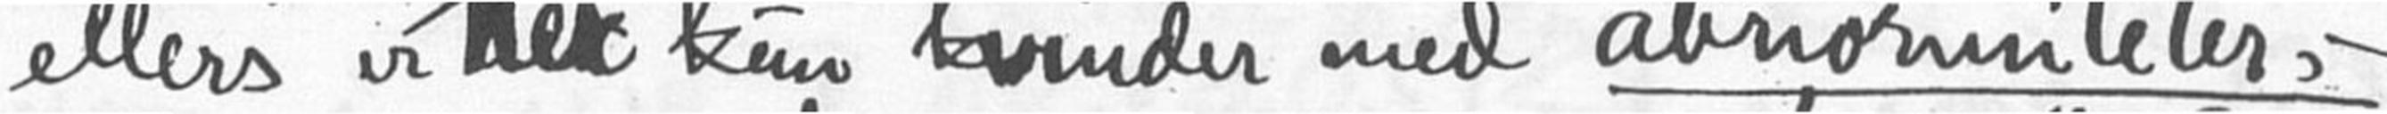ellers er her kun kvinder med abnormiteter, -
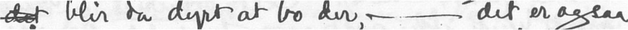det blir da dyrt at bo der, - det er ogsaa
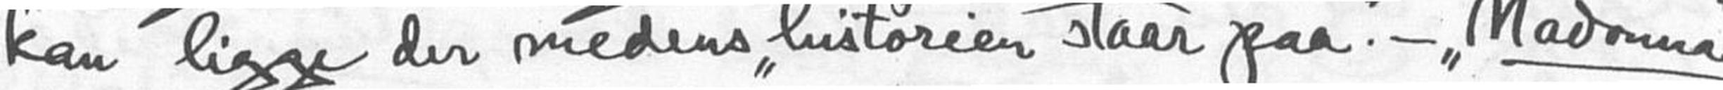kan ligge der medens "historien staar paa". - "Madonna
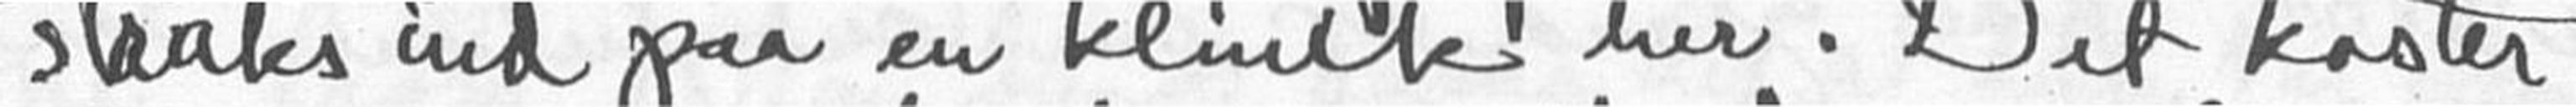straks ind paa en klinik her. Det koster
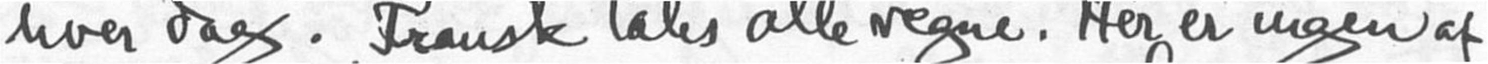hver dag. Fransk tales alle vegne. Her er ingen af
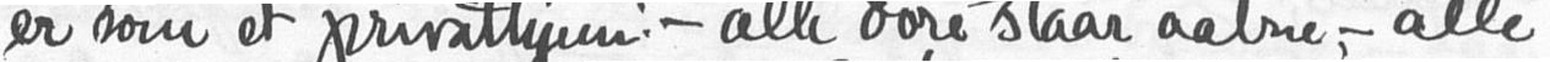er som et privathjem; - alle døre staar aabne, - alle
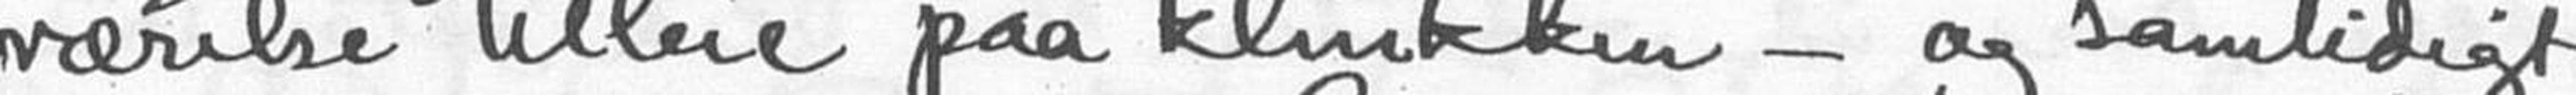værelse tilleie paa klinikken - og samtidigt
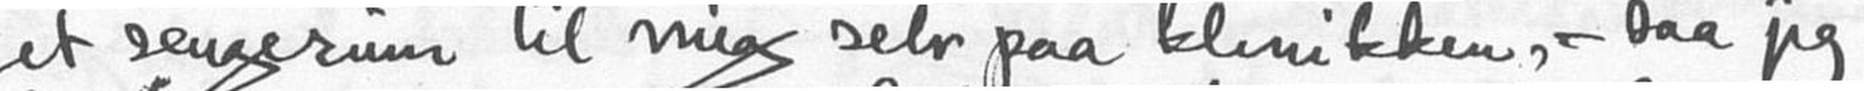et sengerum til mig selv paa klinikken, - saa jeg
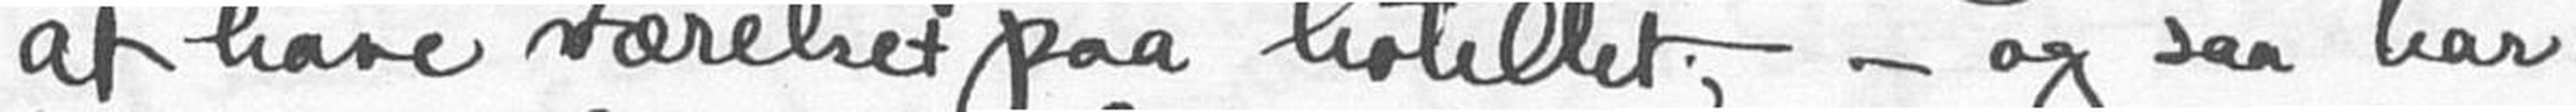at have værelset paa hotellet, - og saa har
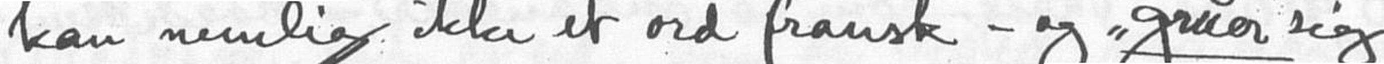kan nemlig ikke et ord fransk - og "gruer" sig
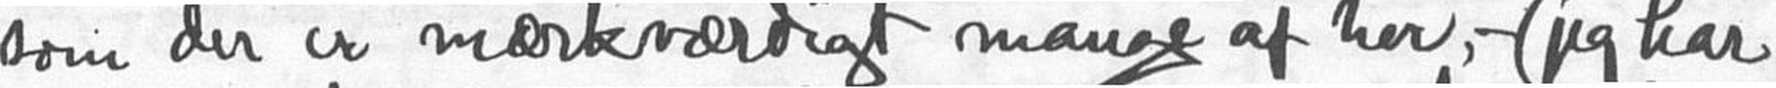som der er mærkværdigt mange af her; - (jeg har
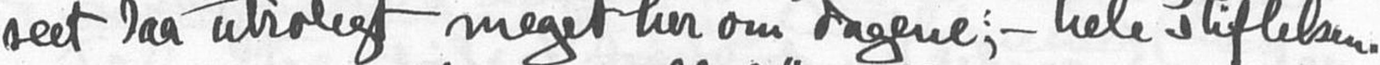seet saa utroligt meget her om dagene; - hele stiftelsen
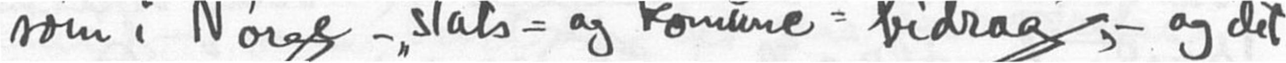som i Norge - "stats- og komune-bidrag",- og det
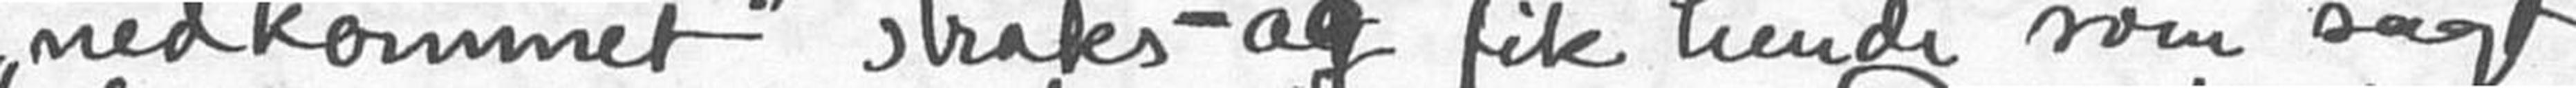"nedkommet" straks - og fik hende som sagt
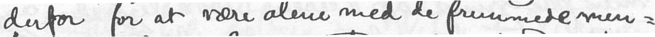derfor for at bære alene med de fremmede men-
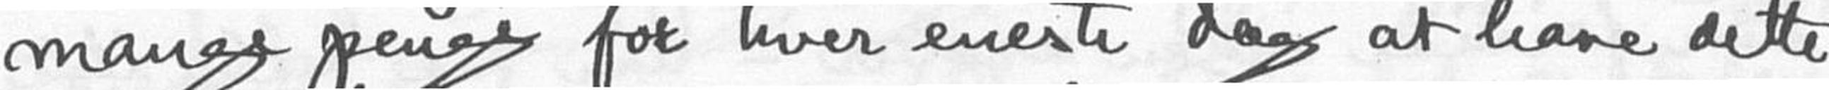mange penge for hver eneste dag at have dette
